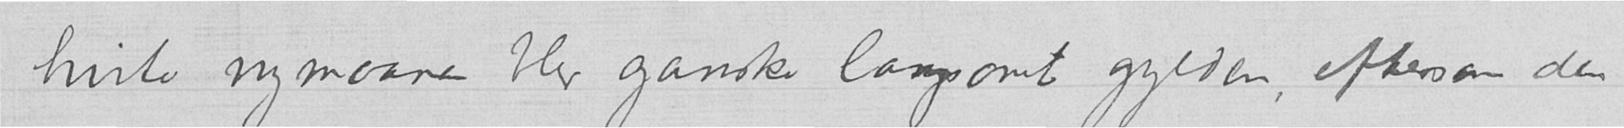hvite nymaanen blev ganske langsomt gylden, eftersom den
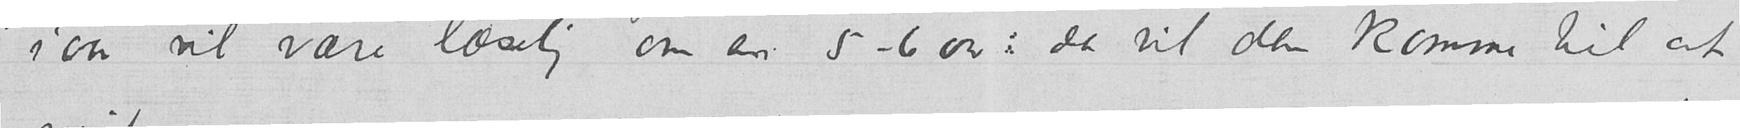iaar vil være læselig om en 5-6 aar: da vil den komme til at
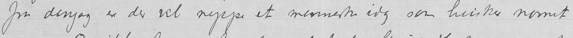fra dengang er der vel neppe et menneske idag som husker navnet
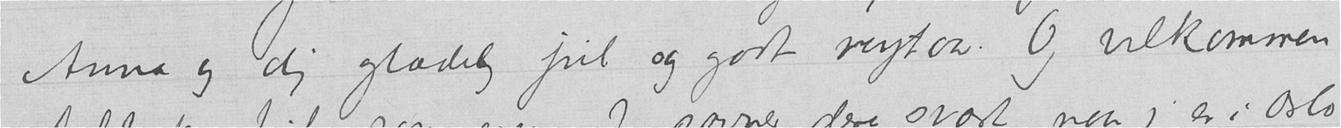Anna og dig glædelig jul og godt nytaar. Og velkommen
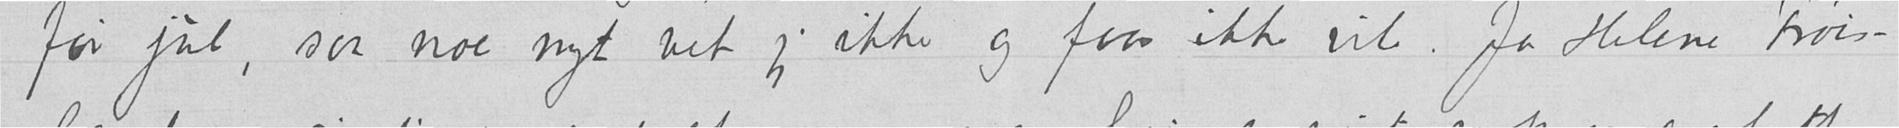før jul, saa noe nyt vet jeg ikke og faar ikke vite. Ja Helene Frøis-
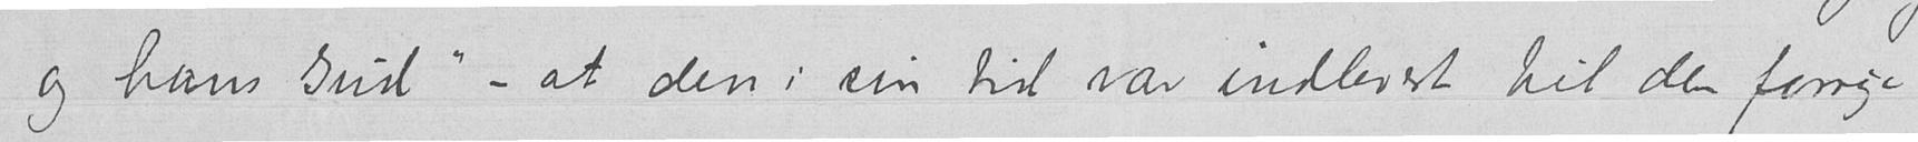og hans Gud" – at den i sin tid var indlevert til den forrige
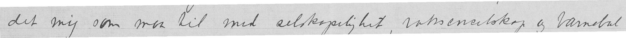det mig som maa til med selskapelighet, voksenselskap og barnebal
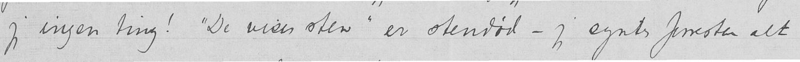jeg ingen ting!  "De vises sten" er stendød – jeg syntes forresten alt
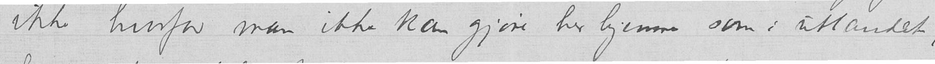ikke hvorfor man ikke kan gjøre her hjemme som i utlandet,
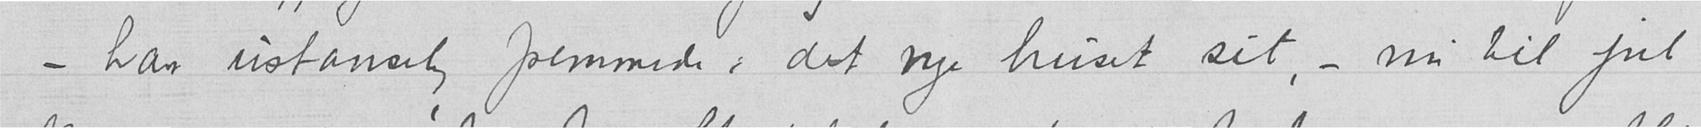– har ustanselig fremmede i det nye huset sit, – nu til jul
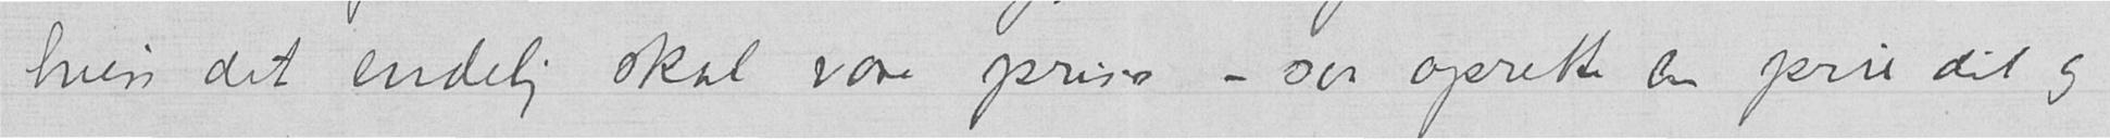hvis det endelig skal være priser – saa oprett en pris dit og
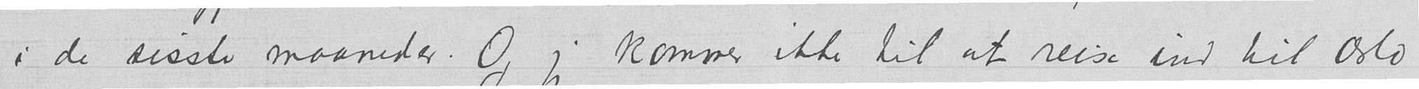i de sisste maaneder. Og jeg kommer ikke til at reise ind til Oslo
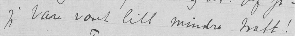jeg bare været litt mindre trætt!
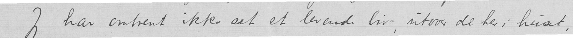Jeg har omtrent ikke set et levende liv, utover de her i huset,
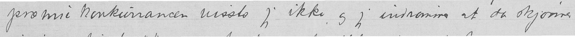præmiekonkurrancen visste jeg ikke, og jeg indrømmer at da skjønner
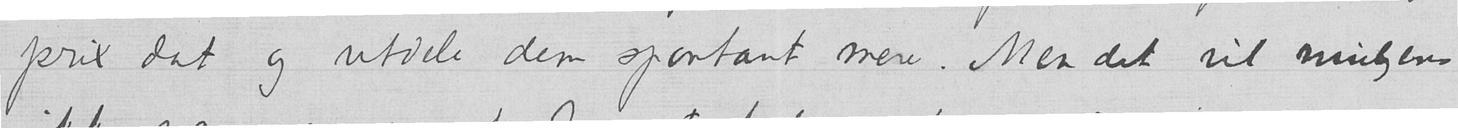pris dat og utdele dem spontant mere. Men det vil muligens
